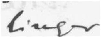linger.
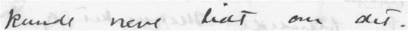kunde være lidt om det.
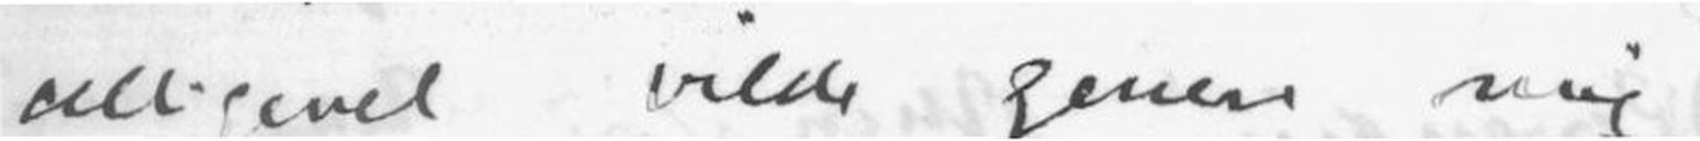alligevel vilde genere mig,
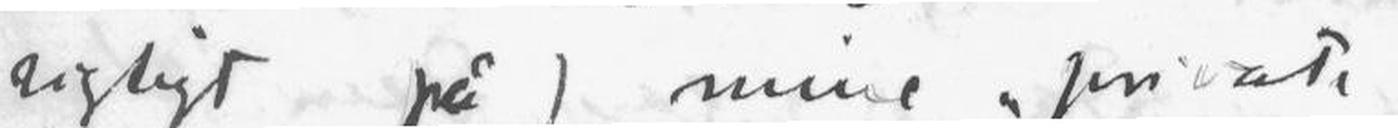rigtigt på) mine private
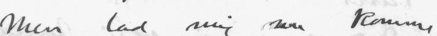Men lad mig nu komme
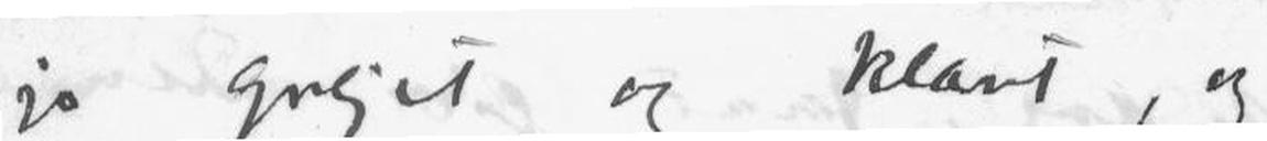jo grejet og klart, og
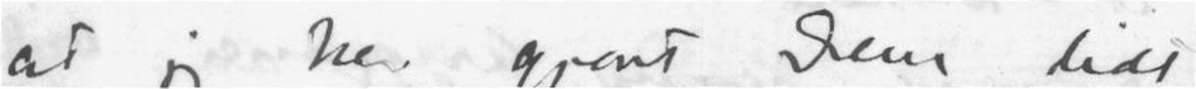at jeg har gjeot Dem lidt
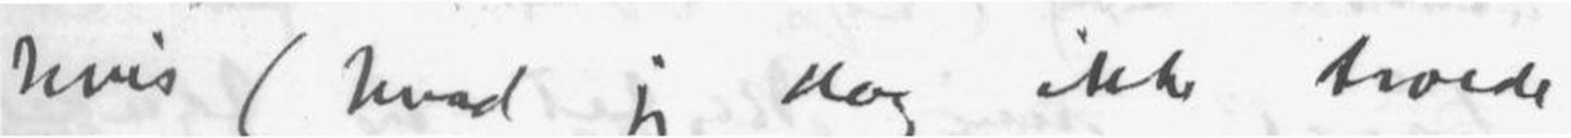hvis (hvad jeg dog ikke troede
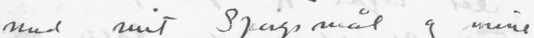med mit Spørgsmål og mine
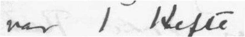var 1 Hefte.
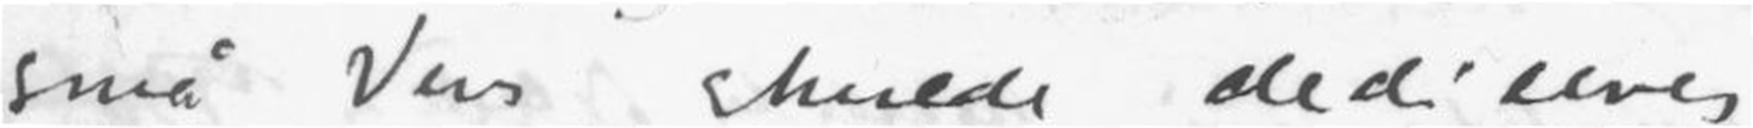små Vers skulde dediceres
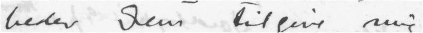beder Dem tilgive mig,
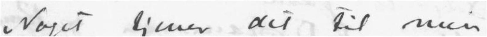Noget tjener det til min
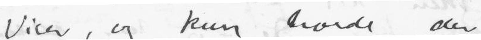Viser, og kun troede der
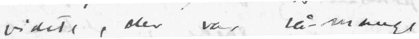vidste, der var så-mange
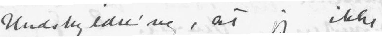Undskyldning, at jeg ikke
